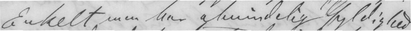Enkelt men har almindelig Gyldighed
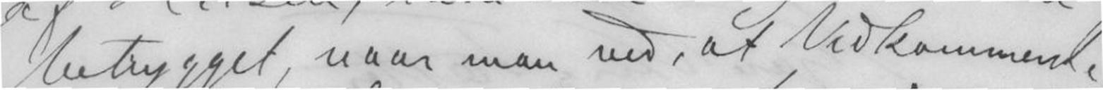betrygget, naar man ved, at Vedkommende
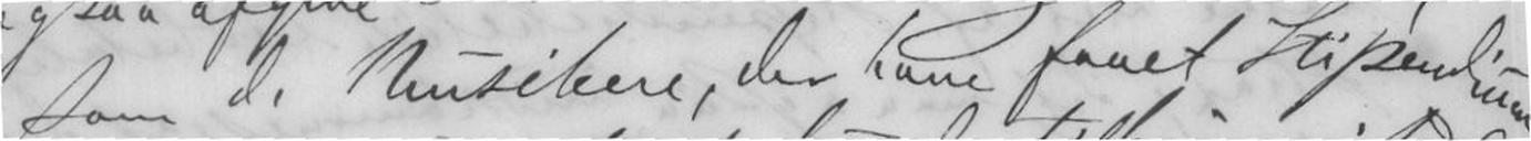som de Musikere, der have faaet Stipendium
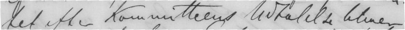tet efter Kommitteens Udtalelse bliver
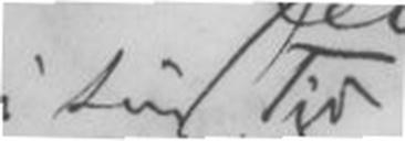i sin Tid
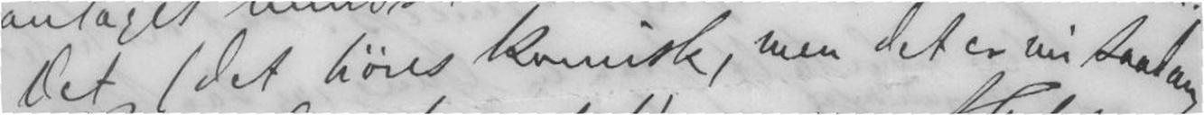det (det høres komisk, men det er nu saadan
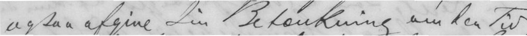ogsaa afgive sin Betænkning om den Tid
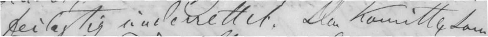feilagtig underrettet. Den Komitte, som
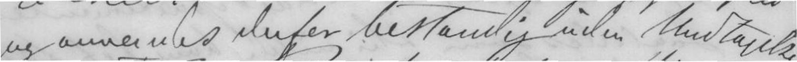og anvendes derfor bestandig uden Undtagelse;
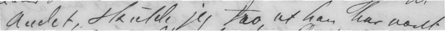Andet, skulde jeg tro at han har været
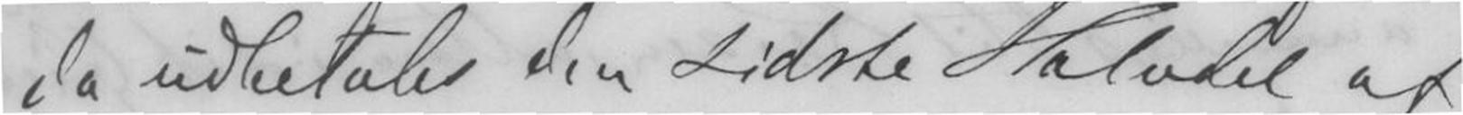da udbetales den sidste Halvdel af
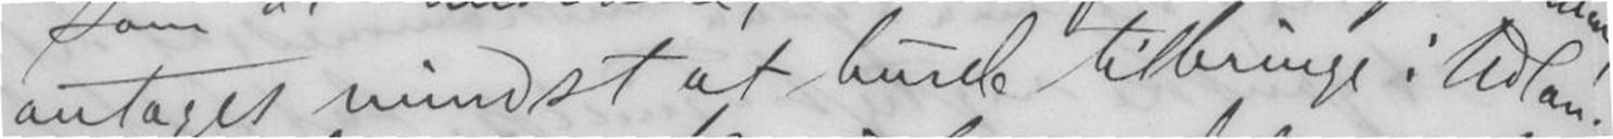antages mindst at burde tilbringe i Udlan-
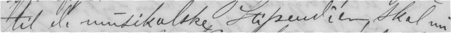til de musikalske Stipendier, skal nu
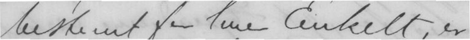bestemt for hver Enkelt, er
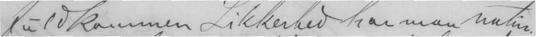fuldkommen Sikkerhed har man natur-
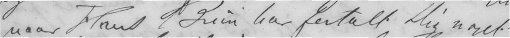naar Hans Brun har fortalt Dig noget
